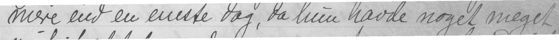mere end en eneste dag, da hun havde noget meget
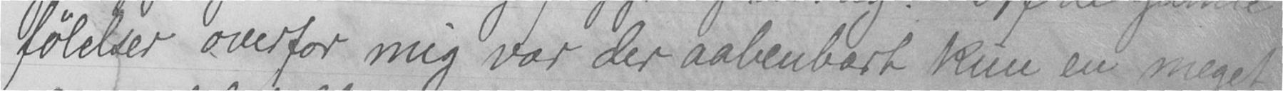følelser overfor mig var der aabenbart kun en meget
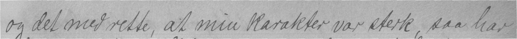og det med rette, at min karakter var sterk, saa har
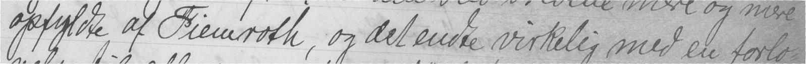opfyldte af Tiemroth, og det endte virkelig med en forlo-
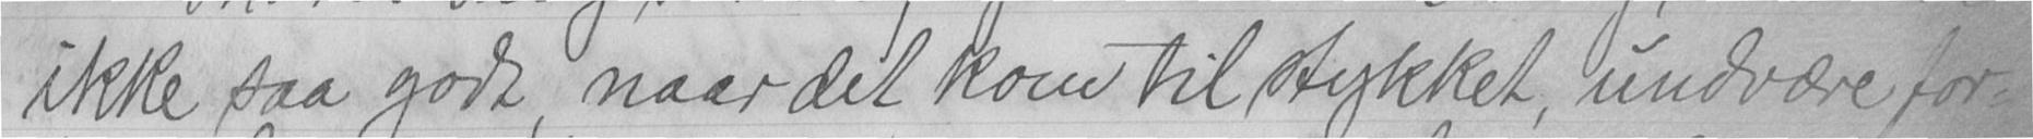ikke saa godt, naar det kom til stykket, undvære for-
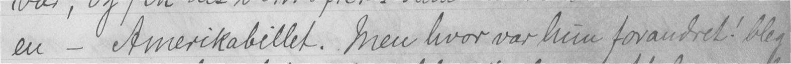en - Amerikabillet. Men hvor var hun forandret! bleg
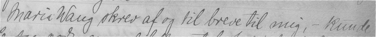Maria Wang skrev af og til breve til mig, - kunde
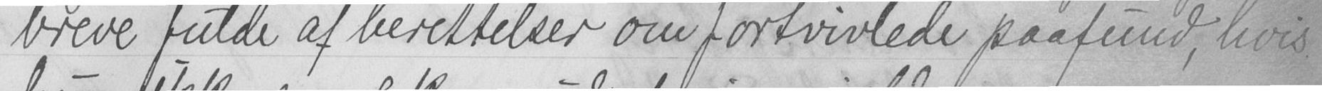breve fulde af berettelser om fortvivlede paafund, hvis
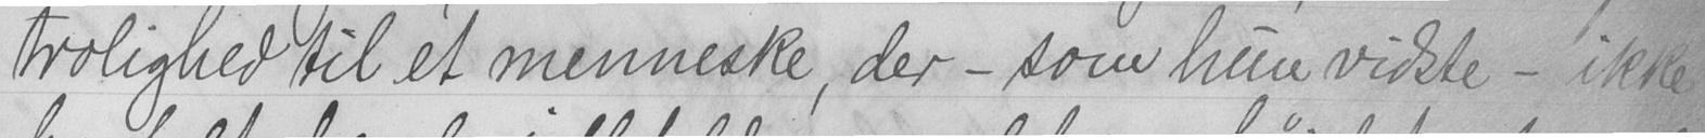trolighed til et menneske, der - som hun vidste - ikke
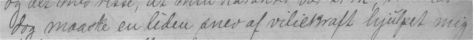dog maaske en liden snev af viliekraft hjulpet mig
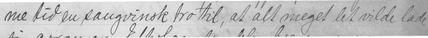me tid en sangvinsk tro til, at alt meget let vide lade
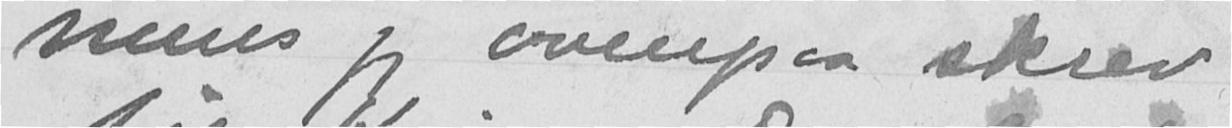mens jeg ovenpaa skrev
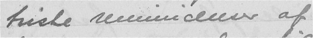triste reminicenser af
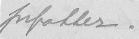forfatter.
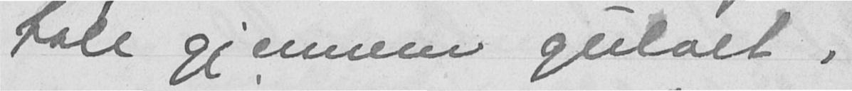tale gjennem gulvet,
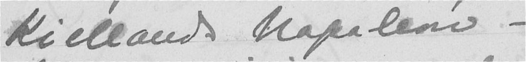Kiellands Napoleon -
